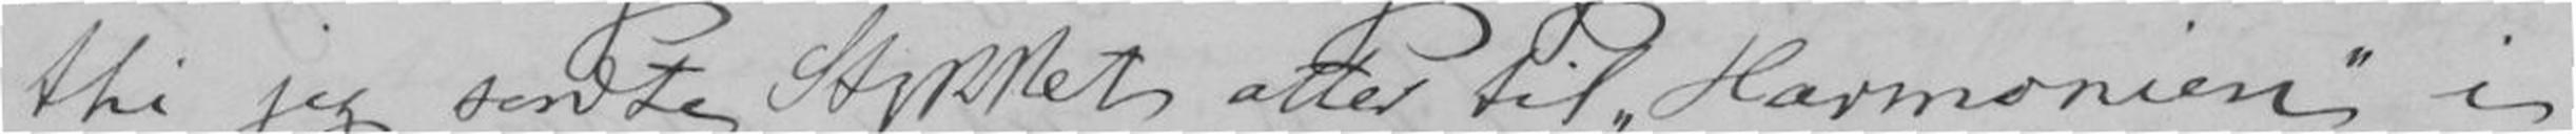thi jeg sendte Stykket atter til Harmonien i
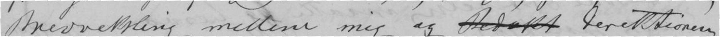Brevveksling mellem mig og Redakt Direktionen;
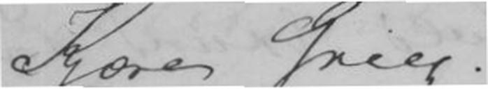Kjære Grieg!
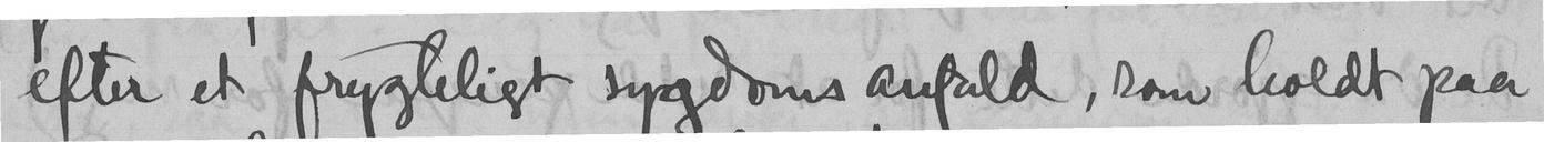efter et frygteligt sygdoms anfald, som holdt paa
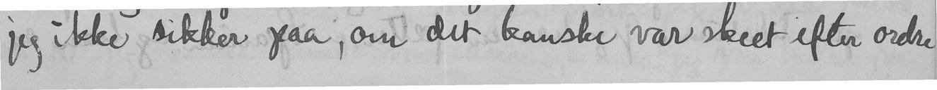jeg ikke sikker paa, om det kanske var skeet efter ordre
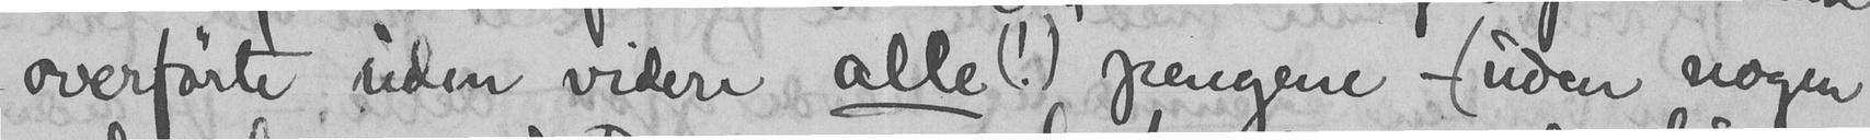overførte uden videre alle (!) pengene - (uden nogen
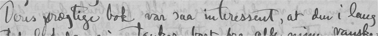Deres prægtige bok var saa interessent, at den i lang
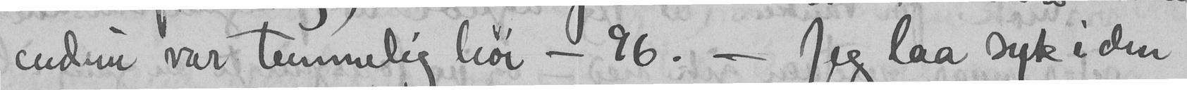endnu var temmelig høi - 96. - Jeg laa syk i den
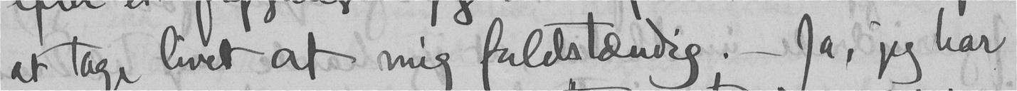at tage livet af mig fuldstændig. - Ja, jeg har
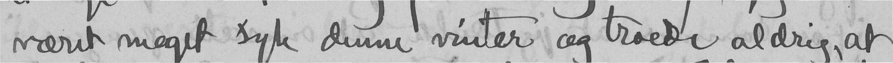været meget syk denne vinter og troede aldrig, at
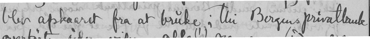blev afskaaret fra at bruke; thi Bergens privatbank
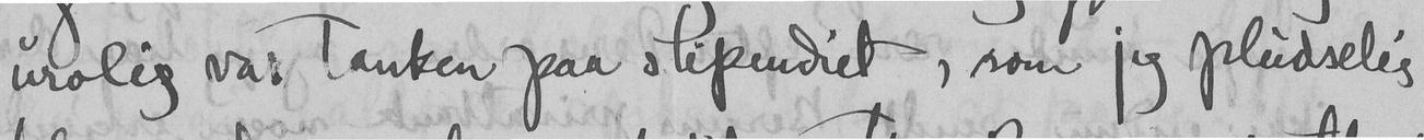urolig var tanken paa stipendiet, som jeg pludselig
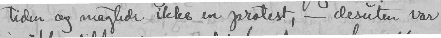tiden og magtede ikke en protest, - desuten var
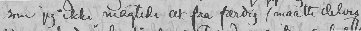som jeg ikke magtede at faa færdig (maatte delvis
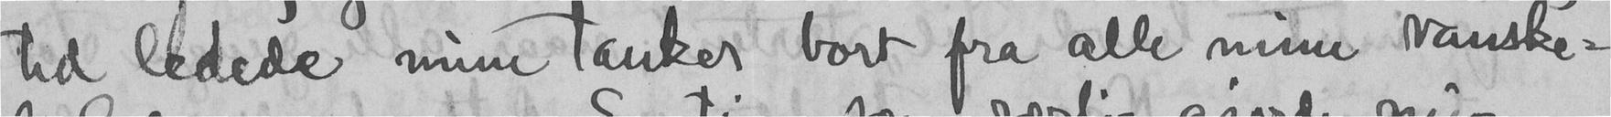tid ledede mine tanker bort fra alle mine vanske-
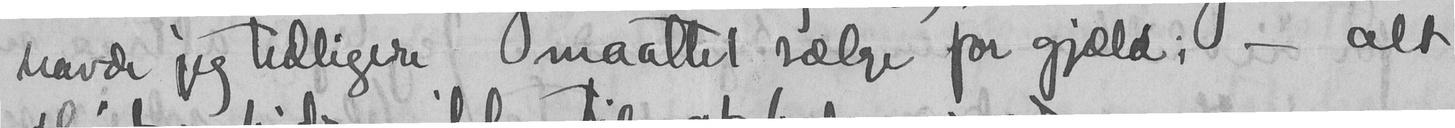havde jeg tidligere maattet sælge for gjæld; - alt
